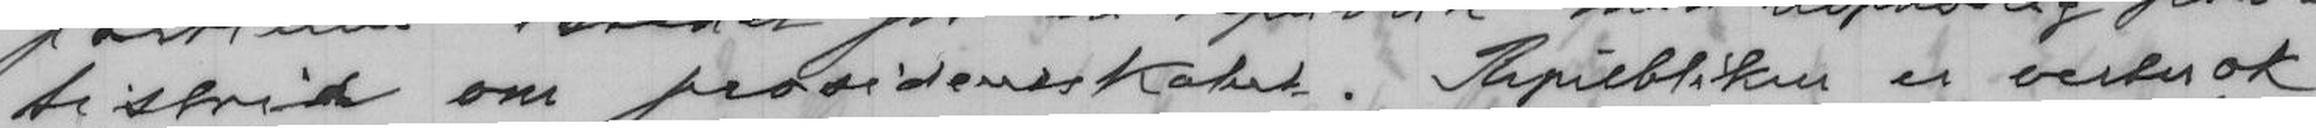tistrid om presidentskabet. Republiken er vistnok
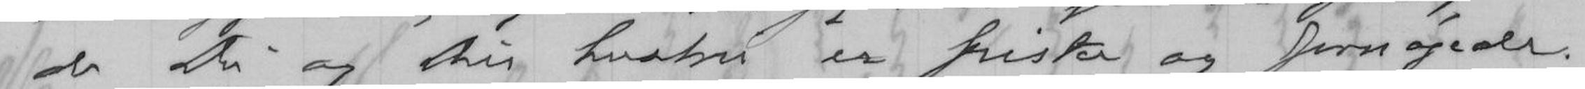de Du og Din hustru er friske og fornøjede.
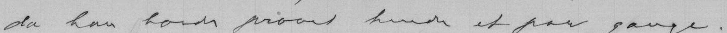da han havde prøvet hende et par gange.
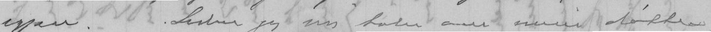igjen. Siden jeg nu taler om mine døttre,
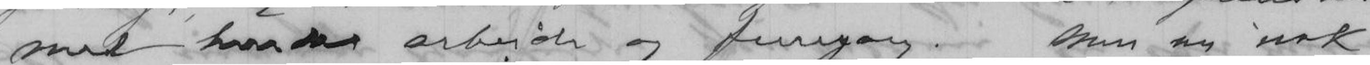med hendes arbeide og fremgang. Men nu nok
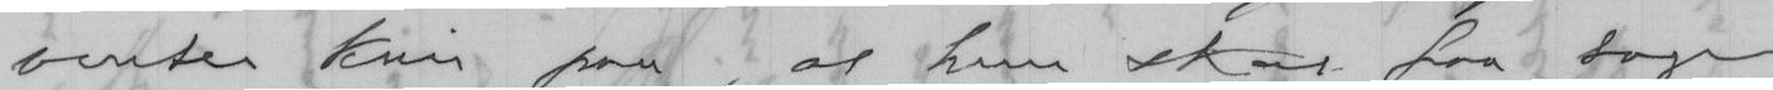venter kun paa, at hun skal faa tage
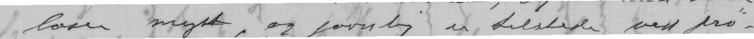læser meget, og jevnlig er tilstede ved prø-
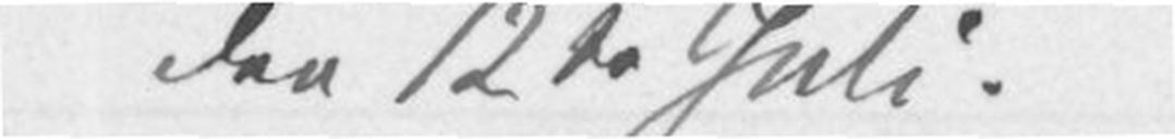den 12te Juli.
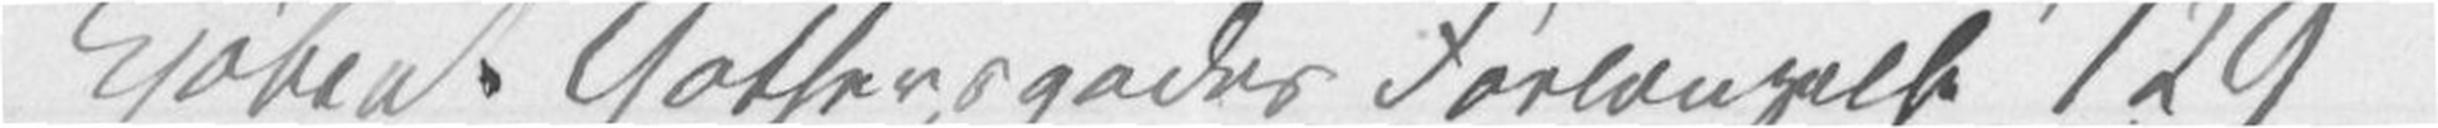Kjøbenh. Gothersgades Forlængelse 129
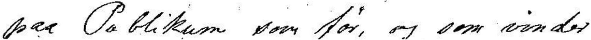paa Publikum som før, og som vinder
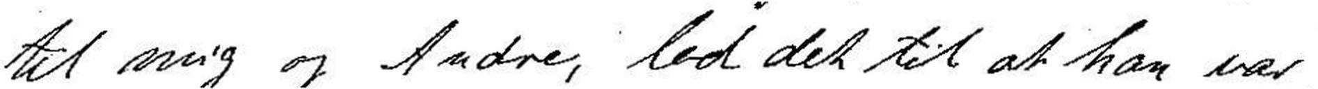til mig og Andre, lod det til at han var
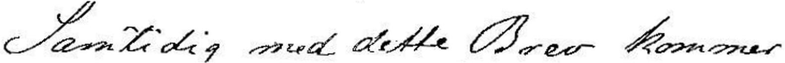Samtidig med dette Brev kommer
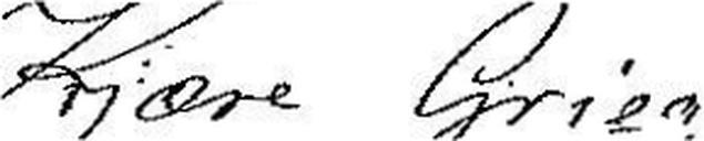Kjære Grieg!
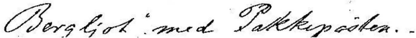Bergljot med Pakkeposten.-
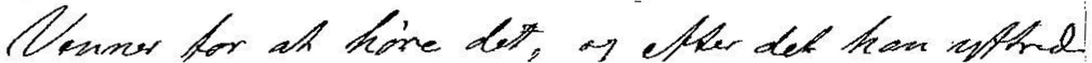Venner for at høre det, og efter det han ytred
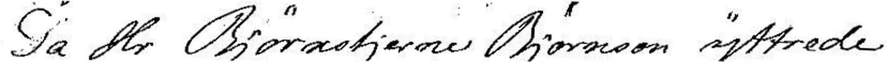Da Hr Bjørnstjerne Bjørnson yttrede
